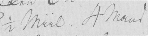1/2 Miil. 4 Mand
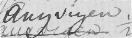Angvigen
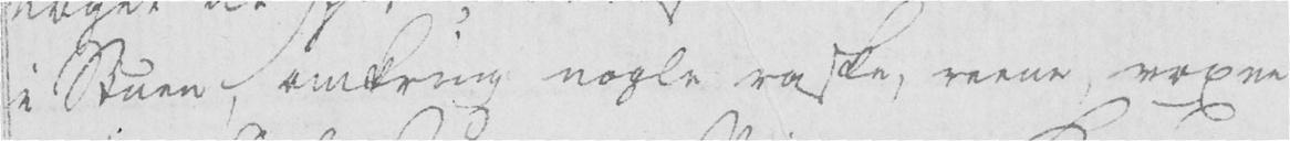i Stuen, omkring nogle raske, reene, voxne
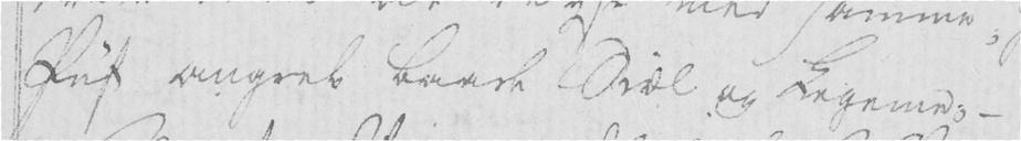Puf angreb baade Siæl og Legeme; -
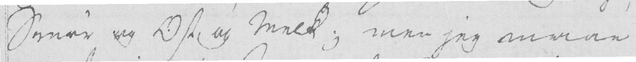Smør og Ost og Melk, men jeg maae
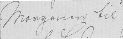Morgenen til
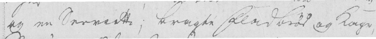og en Serviett; bragte Fladbrød og Kage,
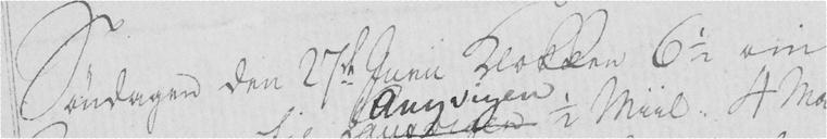Søndagen den 27de Junii Klokken 6 1/2 om
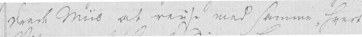drede Miil at reyse med samme, hvert
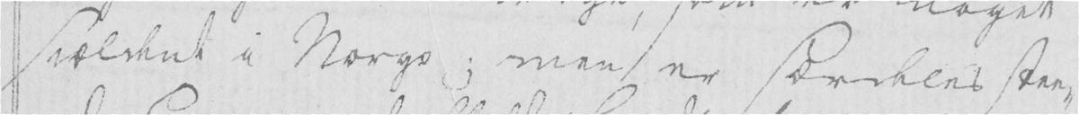siældent i Norge; men er særdeles stee-
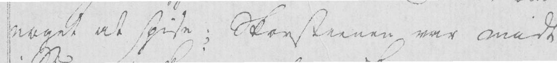noget at spise; Skorsteenen var midt
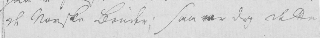de Norske Bønder, saa var dog dette
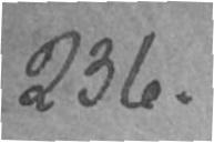236.
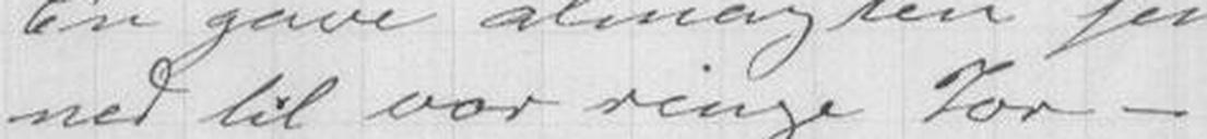ned til vor ringe Jor -
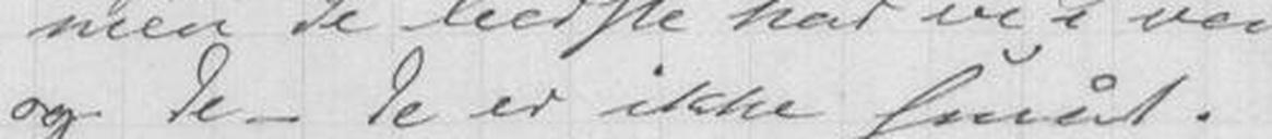og Den De er ikke småt.
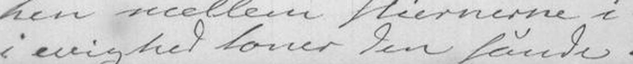i evighed toner den sande.
